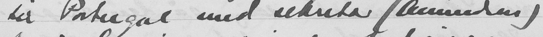til Portugal med sekretær (Amundsen)
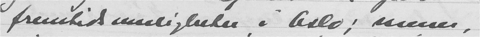fremtidsmuligheter i Oslo; mener,
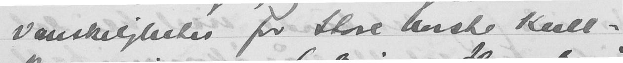vanskeligheter for Store Norske Kull-
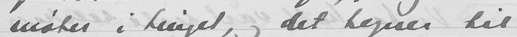møter i tinget, og det tegner til
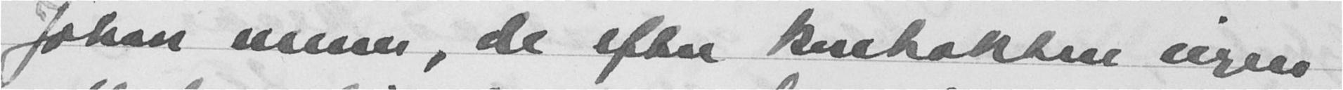Johan mener, de efter kontrakten ingen
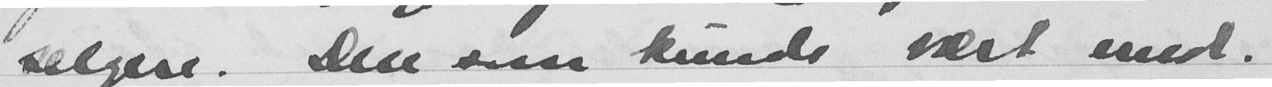selgere. Den som kunde vært med.
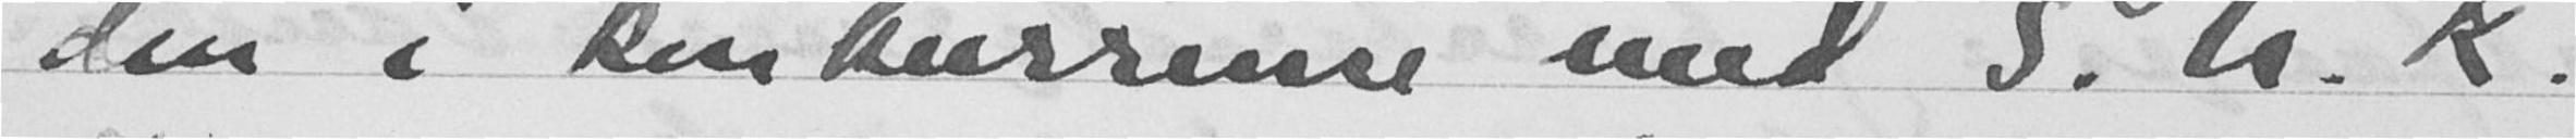den i konkurranse med S. K. K.
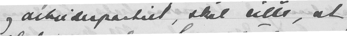og arbeiderpartiet, skal ville, at
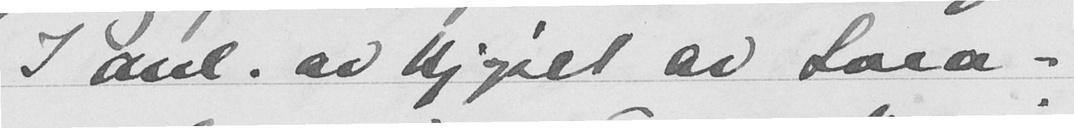I anl. av bygget av Svea-
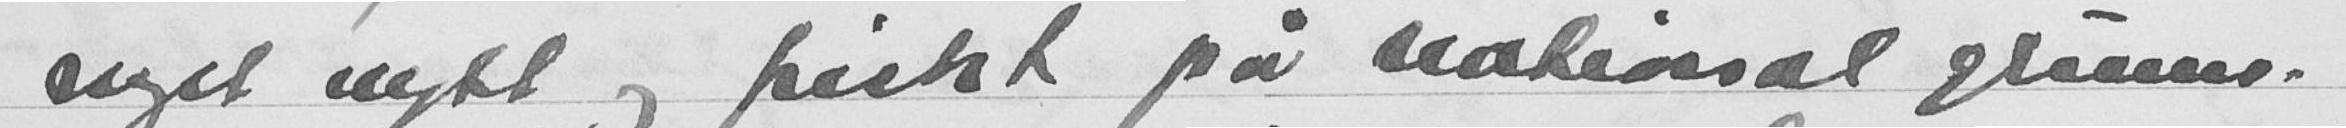noget nytt og friskt på national grunn.
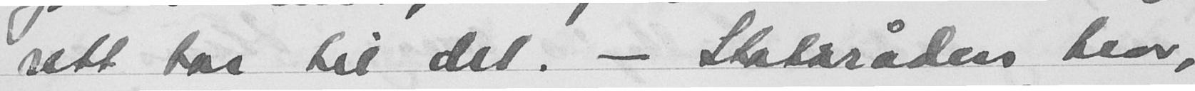rett har til det. - Statsråden tror,
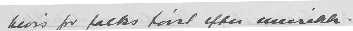bevis for folks tørst efter musikk.
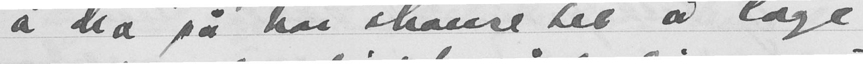å dra på har chanse til å lage
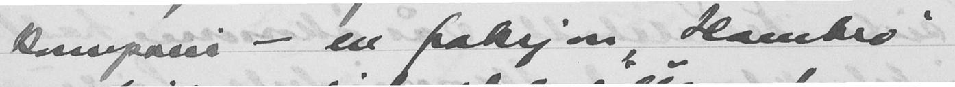kompani - en fraksjon, Hambro
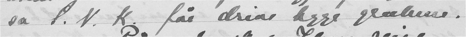sa S. V. K. får drive begge gruvene.
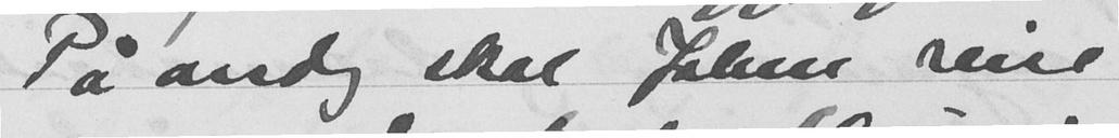På onsdag skal Johan reise
